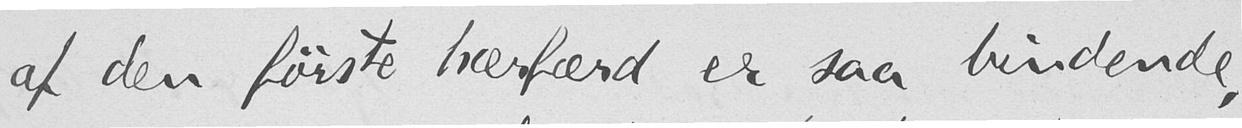af den første hærfærd er saa bindende,
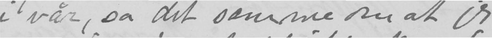i vår, sa det samme om at jeg
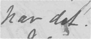har det.
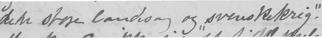den store landsag og "svenskekrig".
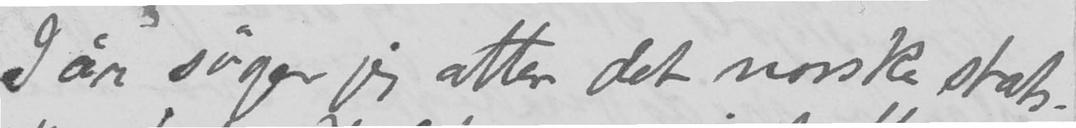I år søger jeg atter det norske stats-
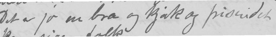Det er jo en bra og kjæk og frisindet
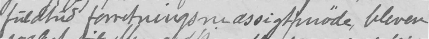fuldtud forretningsmæssigt møde, bleven
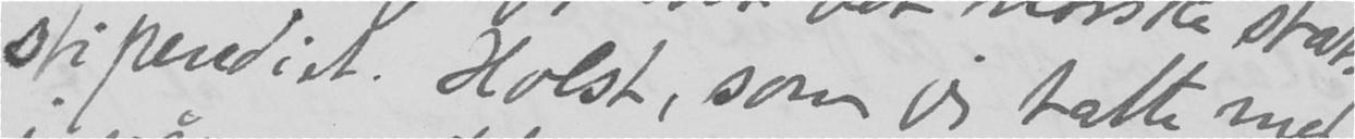stipendiet. Holst, som vi talte med
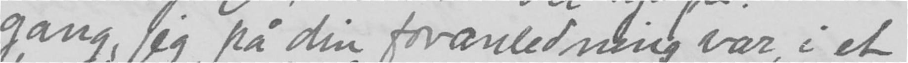gang jeg på din foranledning var, i et
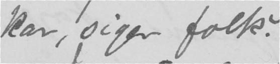kar, siger folk.
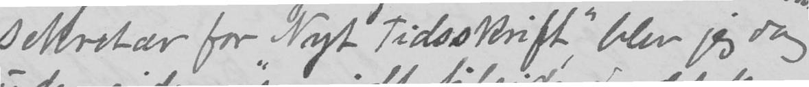sekretær for "Nyt Tidsskrift", blev jeg dog
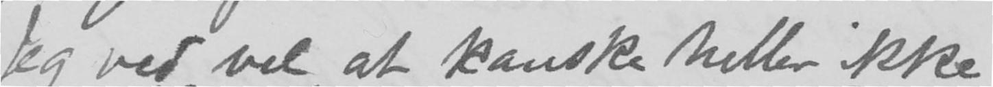Jeg ved vel at kanske heller ikke
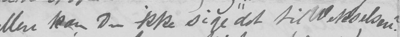Men kan Du ikke sige det til Wekselsen?
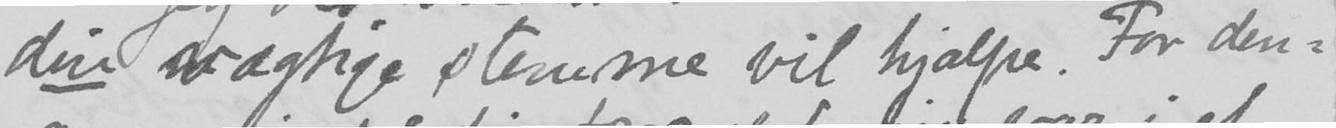din vægtige stemme vil hjælpe. For den-
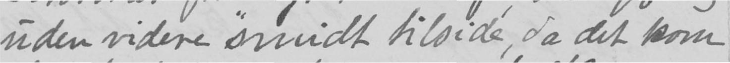uden videre smidt tilside, da det kom
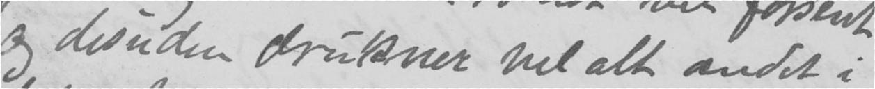og desuden drukner vel alt andet i
